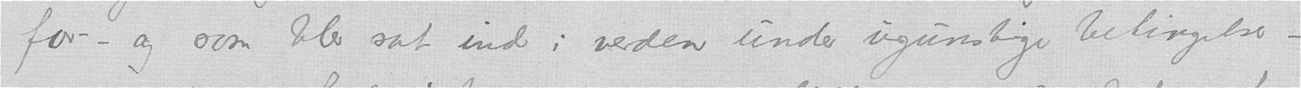for - og som blev sat ind i verden under ugunstige betingelser -
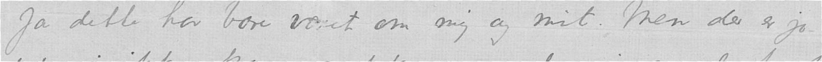Ja dette har bare været om mig og mit. Men der er jo
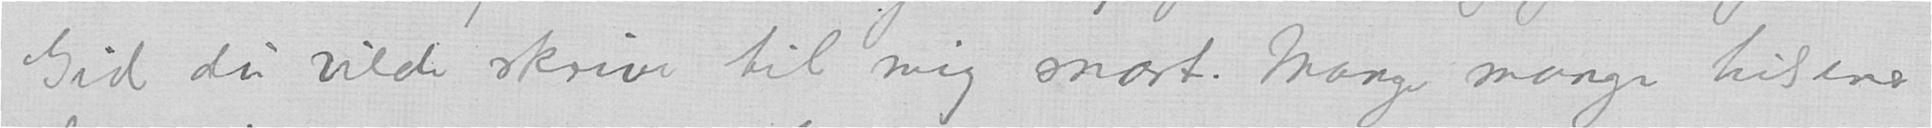Gid du vilde skrive til mig snart. Mange mange hilsener
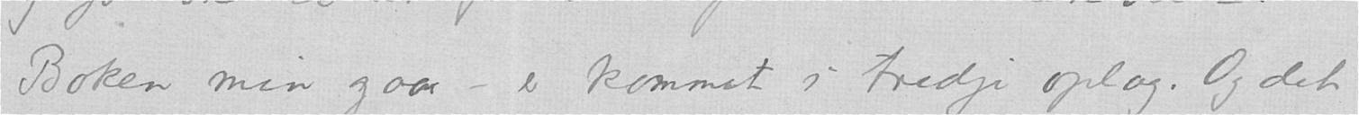Boken min gaar - er kommet i tredje oplag. Og det
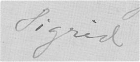Sigrid.
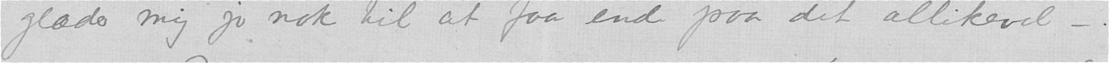glæder mig jo nok til at faa ende paa det allikevel -.
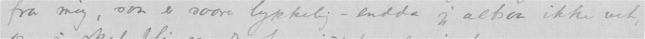fra mig, som er saare lykkelig - endda jeg altsaa ikke vet,
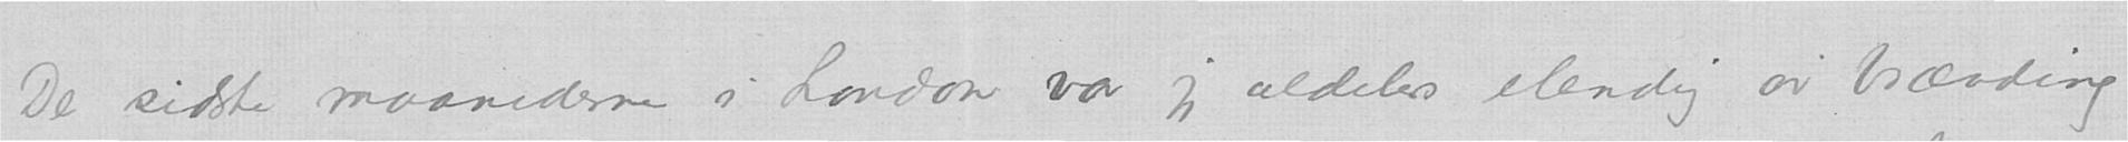De sidste maanederne i London var jeg aldeles elendig av brænding
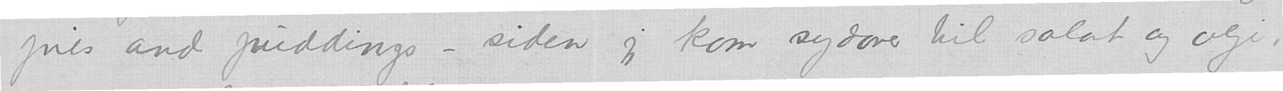pies and puddings - siden jeg kom sydover til salat og olje,
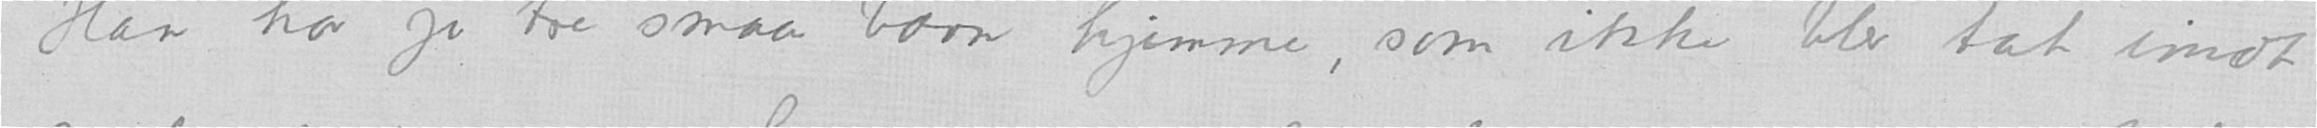Han har jo tre smaa barn hjemme, som ikke blev tat imot
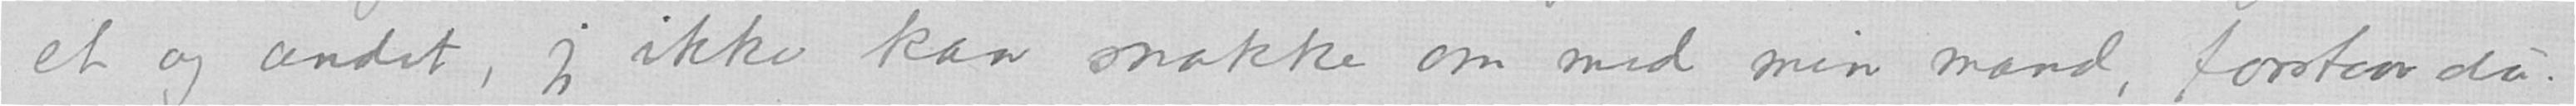et og andet, jeg ikke kan snakke om med min mand, forstaar du.
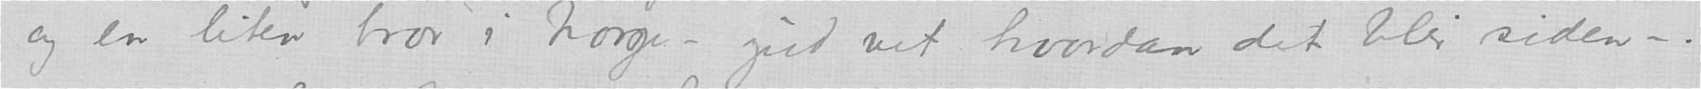og en liten bror i Norge - gud vet hvordan det blir siden -.
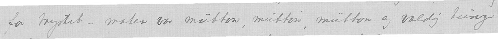for brystet - maten var mutton, mutton, mutton og vældig tunge
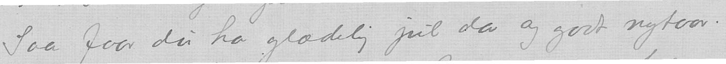Saa faar du har glædelig jul da og godt nytaar.
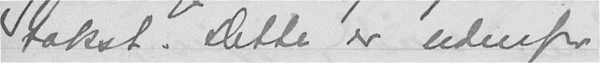takst. Dette er udenfor
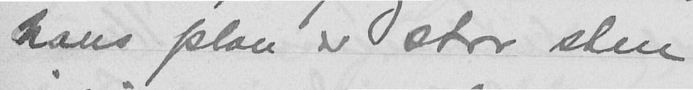hans plan en stor sten
